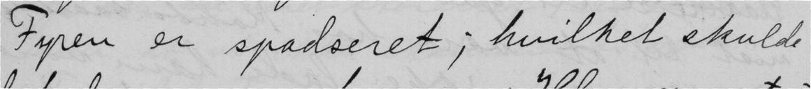Fyren er spadseret; hvilket skulde
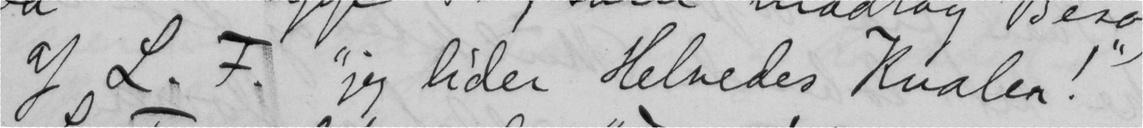af L. F. "jeg lider Helvedes Kvaler!"
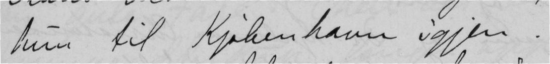hun til Kjøbenhavn igjen.
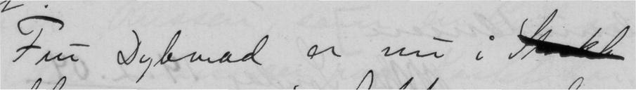Fru Dybwad er nu i
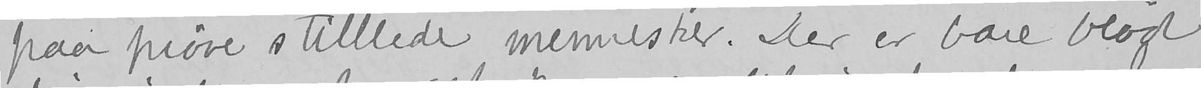paa prøve stilllede mennesker. Der er bare blød
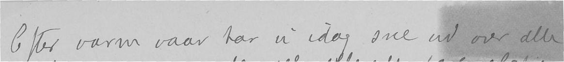Efter varm vaar har vi idag sne ud over alle
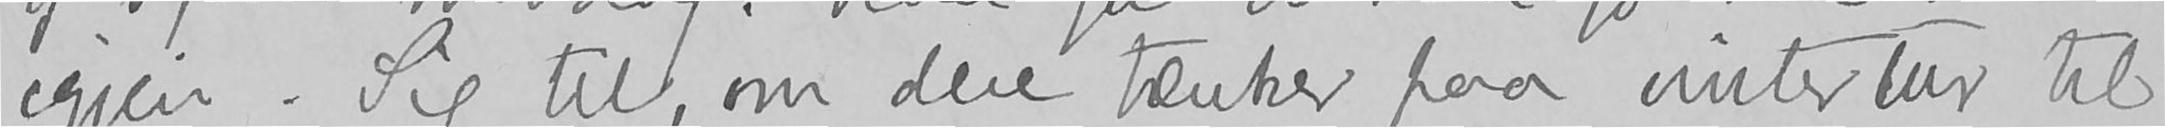igjen. Sig til, om dere tænker paa vintertur til
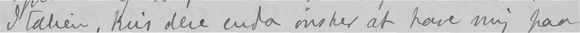Italien, hvis dere enda ønsker at have mig paa
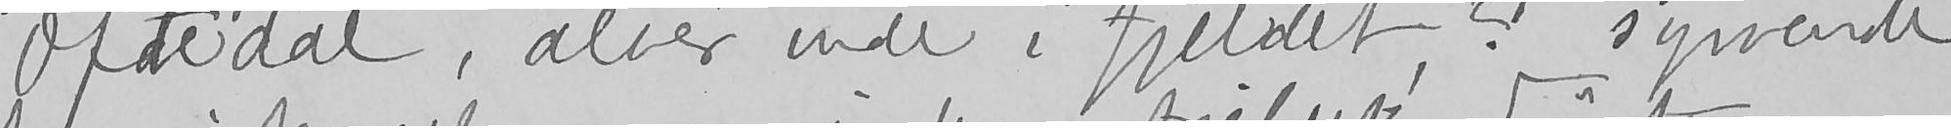Oftedal, alver inde i fjeldet? syvende
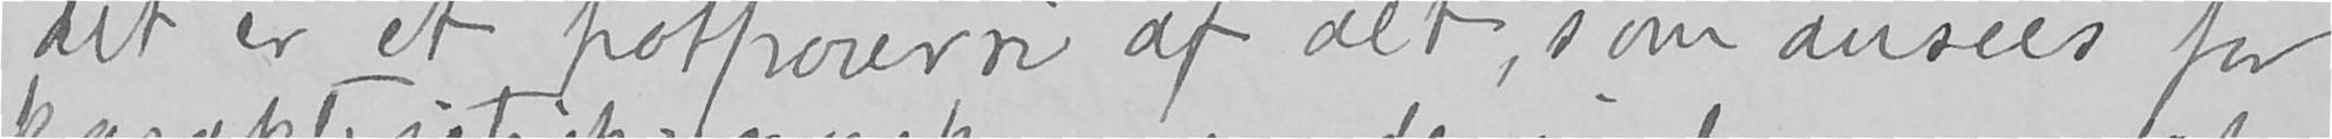det er et potpourri af alt, som ansees for
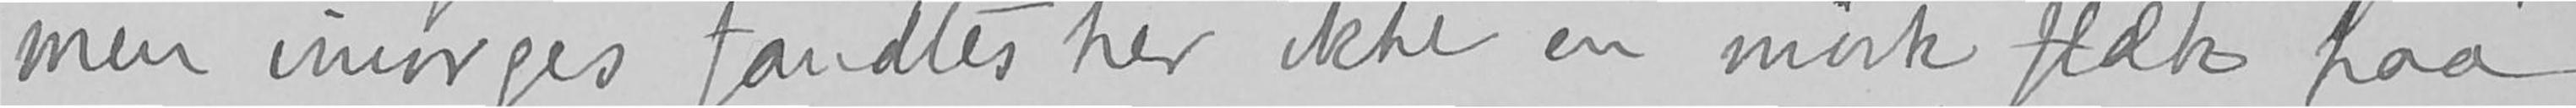men imorges fandtes her ikke en mørk flæk paa
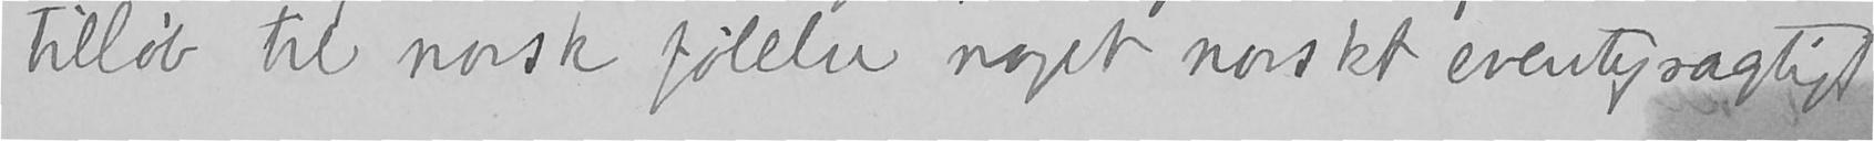tilløb til norsk følelse noget norskt eventyragtigt
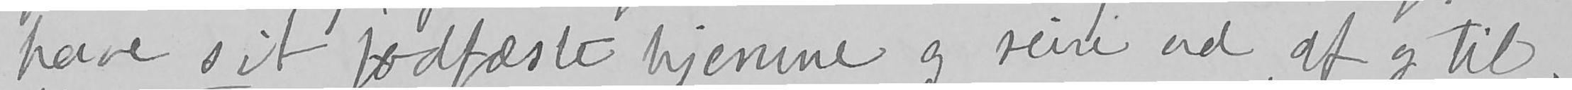have sit fodfæste hjemme og reise ud af og til.
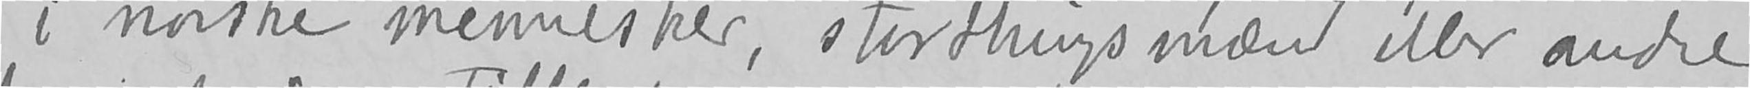i norske mennesker, storthingsmænd eller andre
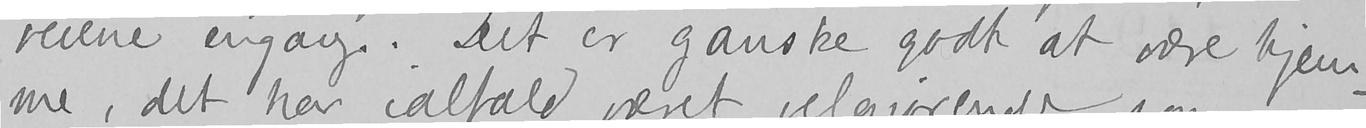veiene engang. Det er ganske godt at være hjem-
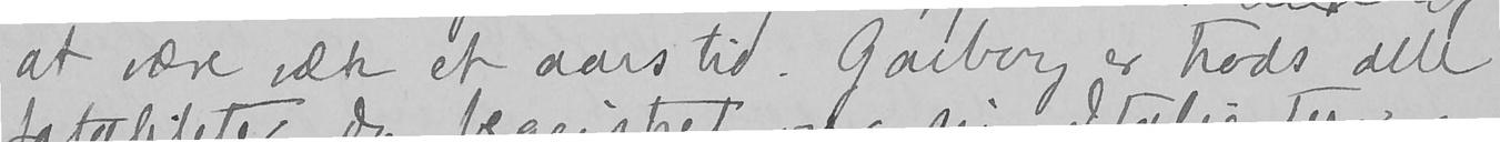at være væk et aars tid. Garborg er trods alle
